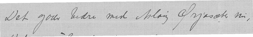Det gaar bedre med Aslaug Ørjasæter nu,
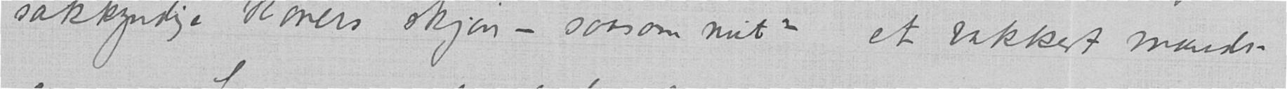sakkyndige koners skjøn - saasom mit - et vakkert mands-
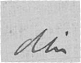din
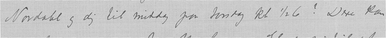Nordahl og dig til middag paa torsdag kl. ½ 6? Dere kan
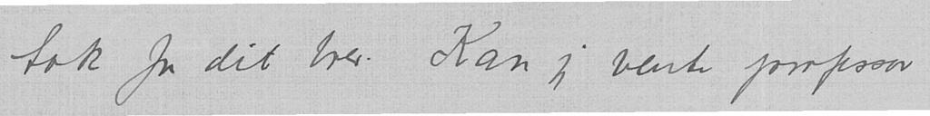tak for dit brev. Kan jeg vente professor
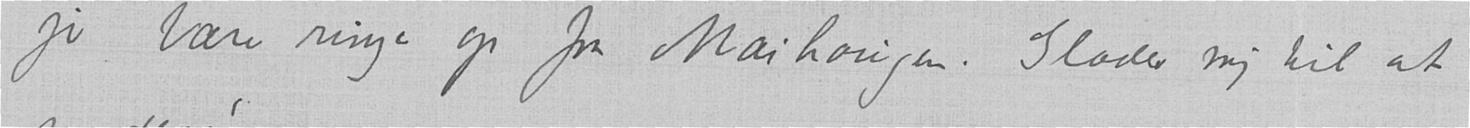jo bare ringe op fra Maihaugen. Glæder mig til at
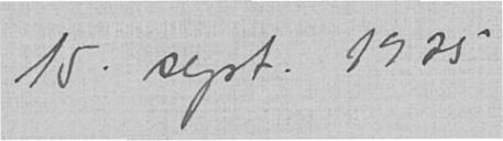15. sept. 1925
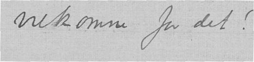velkomne for det!
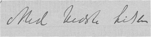Med bedste hilsen
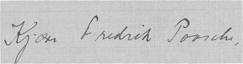Kjære Fredrik Paasche,
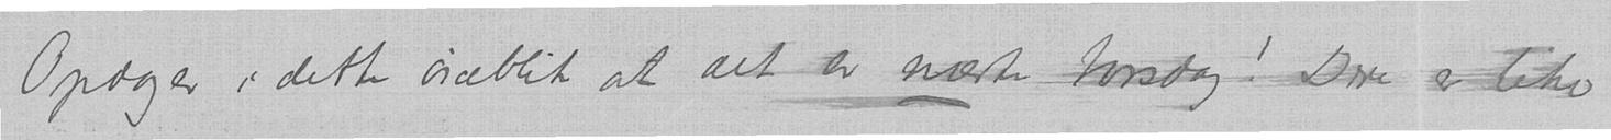Opdager i dette øieblik at det er næste torsdag! Dere er like
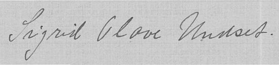Sigrid Olave Undset.
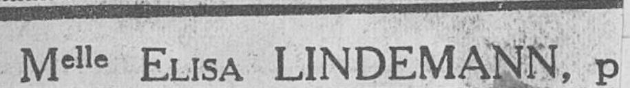Melle ELISA LINDEMANN, p
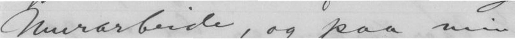Murarbeide, og paa min
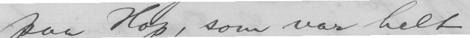paa Hop, som var helt
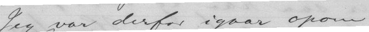Jeg var derfor igaar opom
